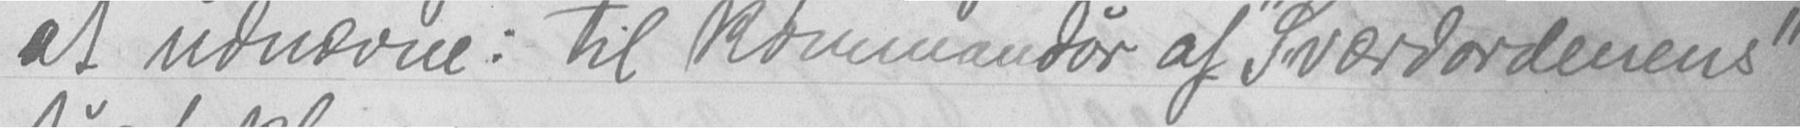at udnævne: til kommandør af "Sværdordenens"
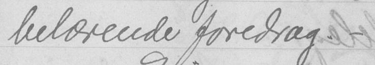belærende foredrag. -
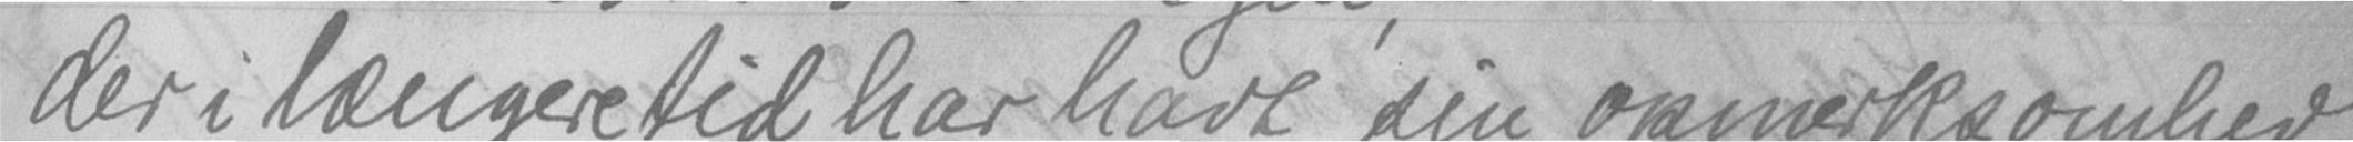der i længere tid har havt sin opmerksomhed
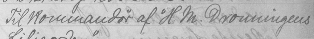Til kommandør af "H. M. Dronningens
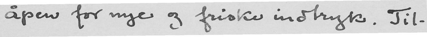åpen for nye og friske indtryk. Til-
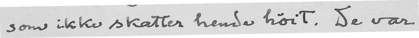som ikke skatter hende høit. De var
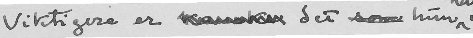Viktigere er kanske det som hun
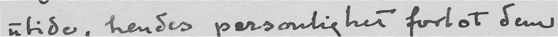utide, hendes personlighet forlot dem
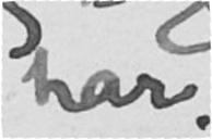har
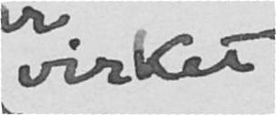virket
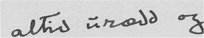altid urædd og
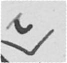i
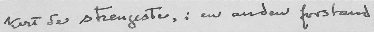kert de strengeste, i en anden forstand
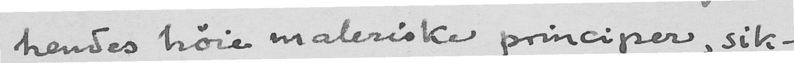hendes høie maleriske principer, sik-
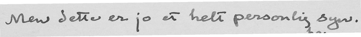Men dette er jo et helt personlig syn.
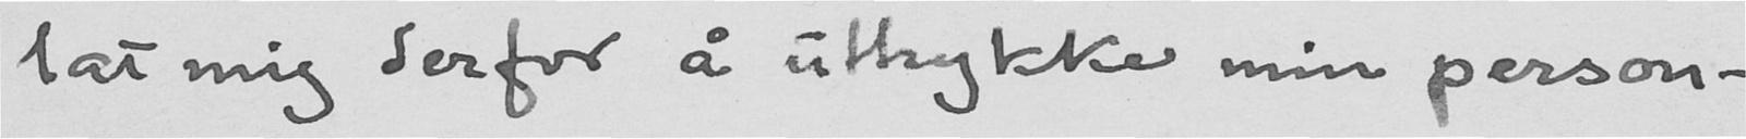lat mig derfor å uttrykke min person-
